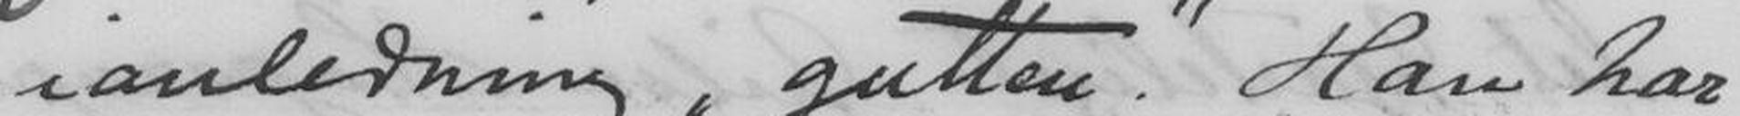ianledning gutten Han har
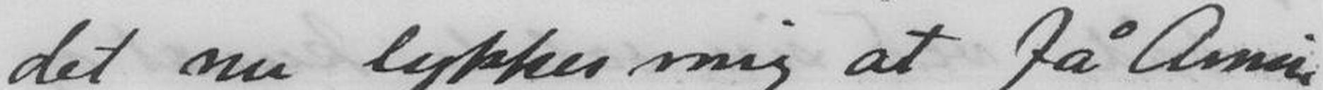det nu lykkes mig at få Annie
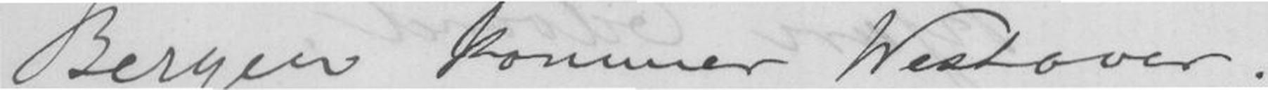Bergen kommer Westover.
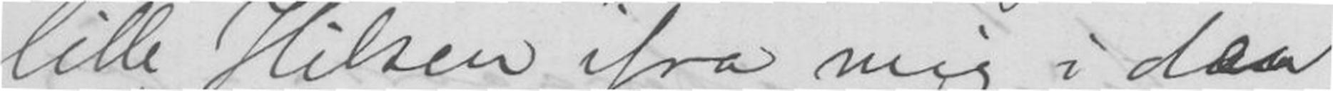lille Hilsen ifra mig i den
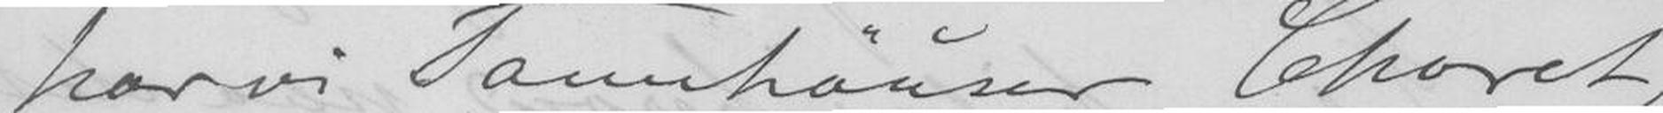har vi Tannhäuser Choret,
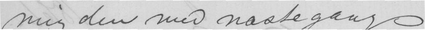mig den med næstegang
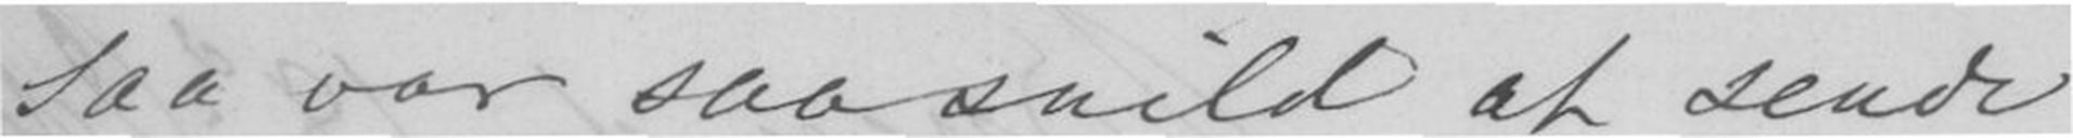saa vær saa snild at sende
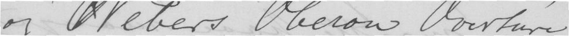og Webers Oberon Overture
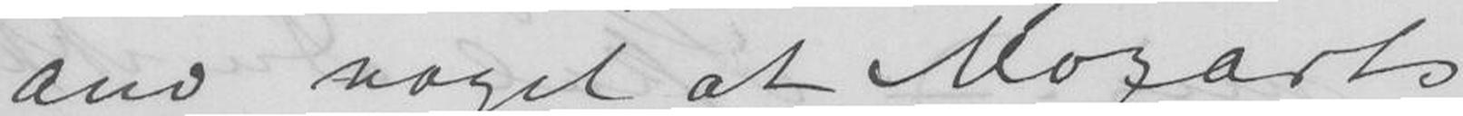ano noget at Mozarts
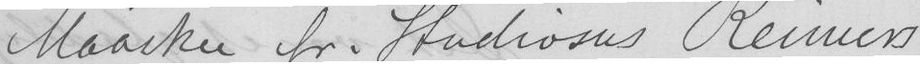Maaske hr. Studiosus Reimers
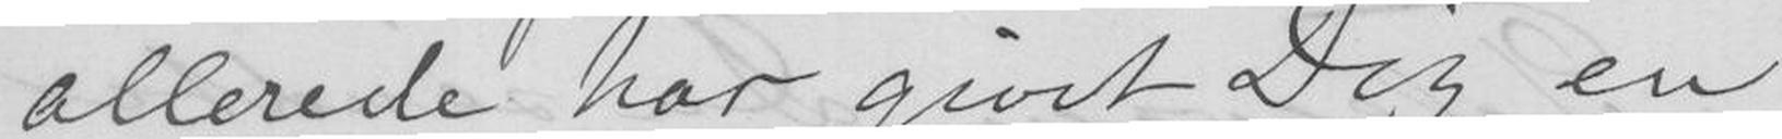allerede har givet Dig en
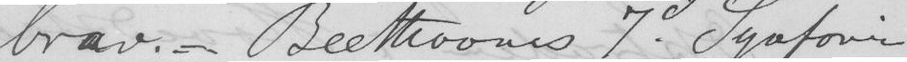brav. - Beethovens 7d Synfoni.
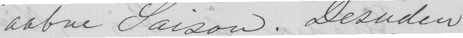aabne Saison. Desuden
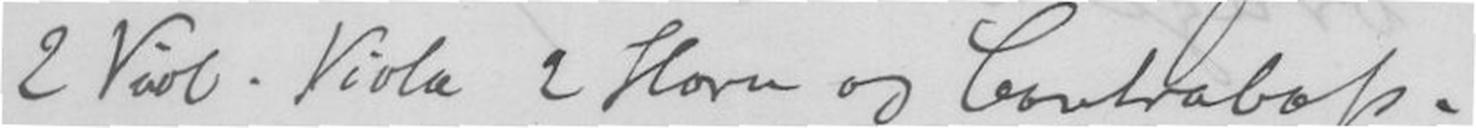2 Viol. Viola 2 Horn og Contrabass.
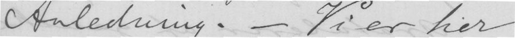Anledning. - Vi er her
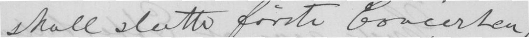skall slutte første Concerten,
